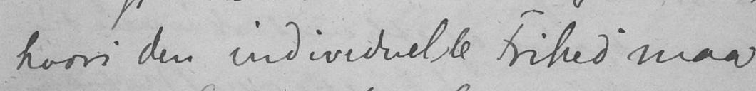hvori den individuelle Frihed maa
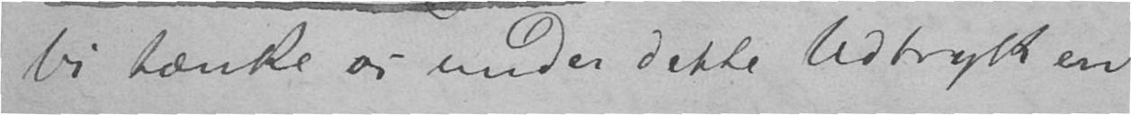Vi tænke os under dette Udtryk en
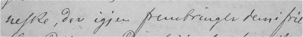neske, der igjen frembringer dem i frie
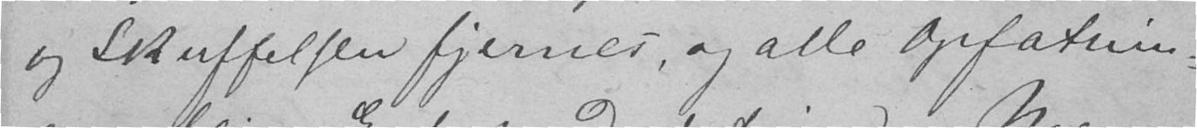og Skuffelsen fjernes, og alle Opfatnin-
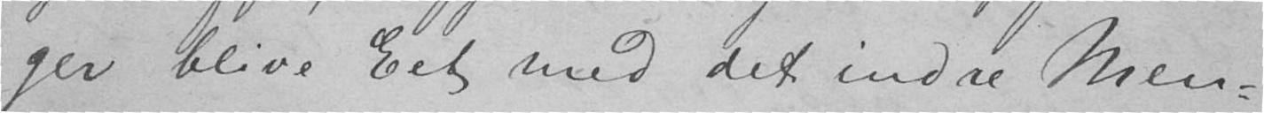ger blive Eet med det indre Men-
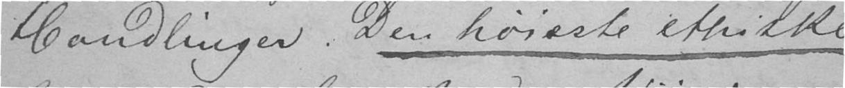Handlinger. Den høieste ethiske
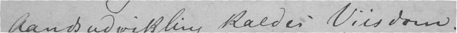Aandsudvikling kaldes Viisdom.
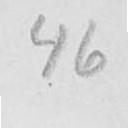46
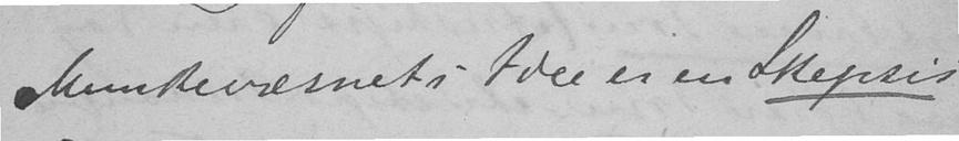Munkevæsnets Idee er en Skepsis
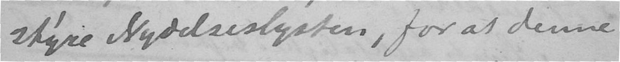styre Nydelseslysten, for at denne
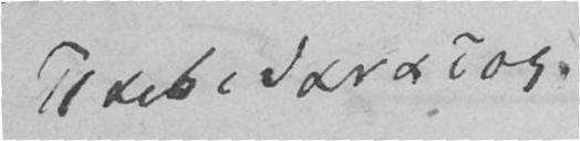gresk.
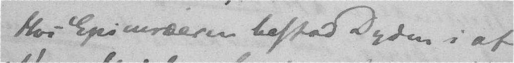Hos Epicuræeren bestod Dyden i at
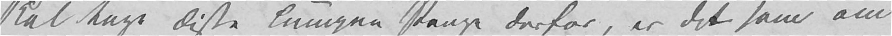skal tage disse lumpne Penge derfor, er det som om
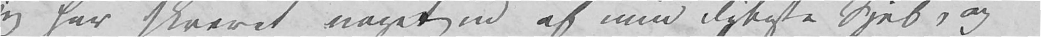jeg har skrevet noget ud af min dybeste Sjel, og
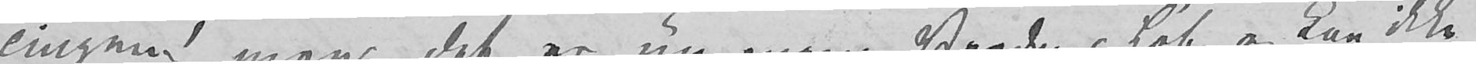Tingen! men det er nu engang Verdens Løb og kan ikke
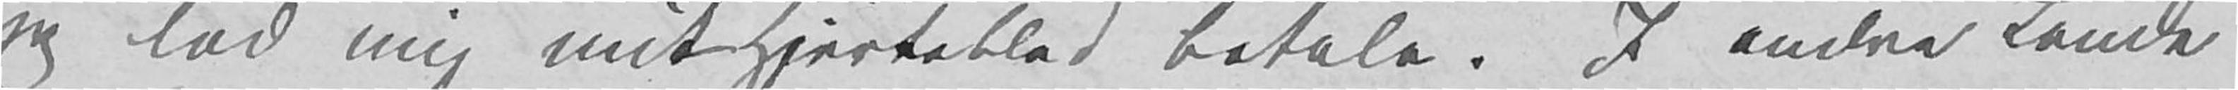jeg lod mig mit Hjerteblod betale. I andre Lande
